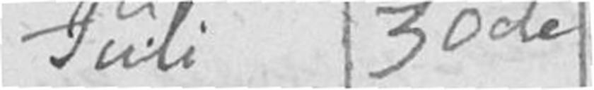Juli 30de
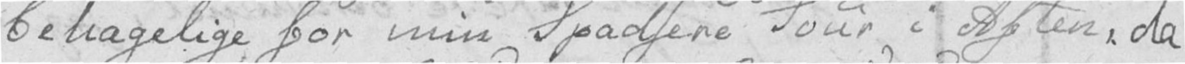behagelige for min Spadsere Tour i Aften, da
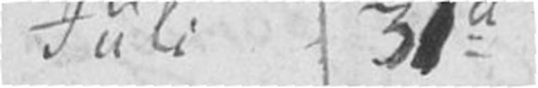Juli 31d
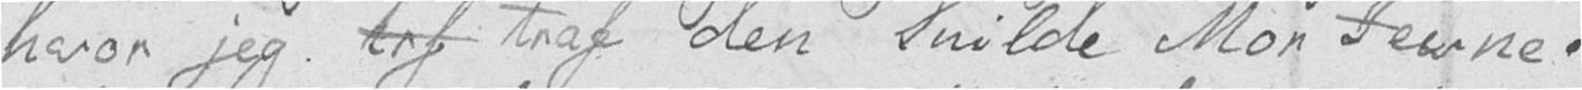hvor jeg trf traf den Snilde Mor Jevne.
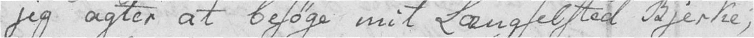jeg agter at besøge mit Længselsted Bjerke,
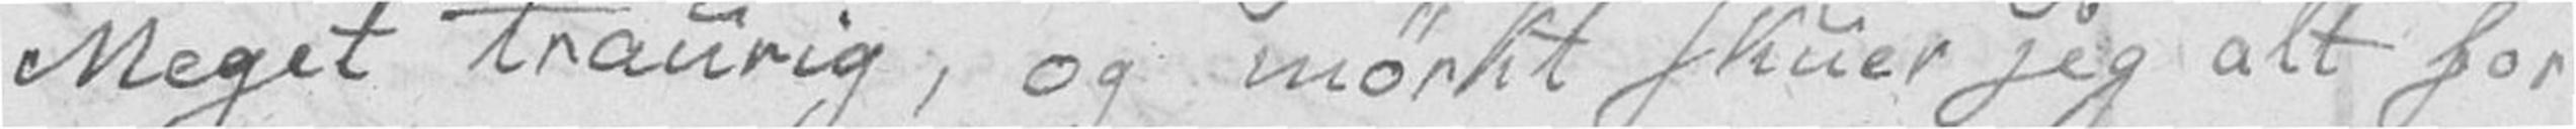Meget traurig, og mørkt skuer jeg alt for
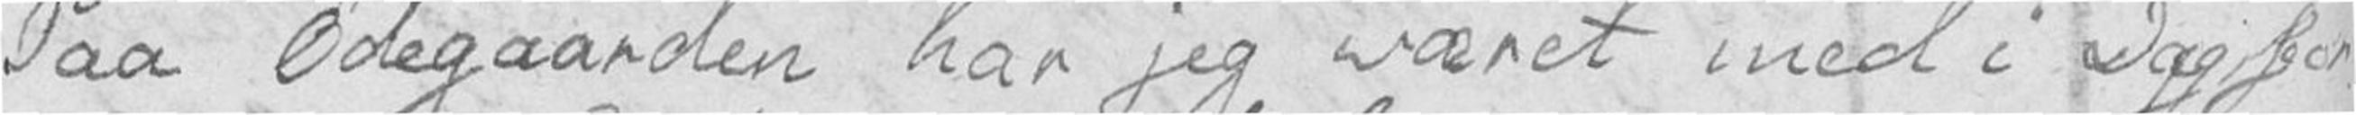Paa Ødegaarden har jeg været med i Dag for
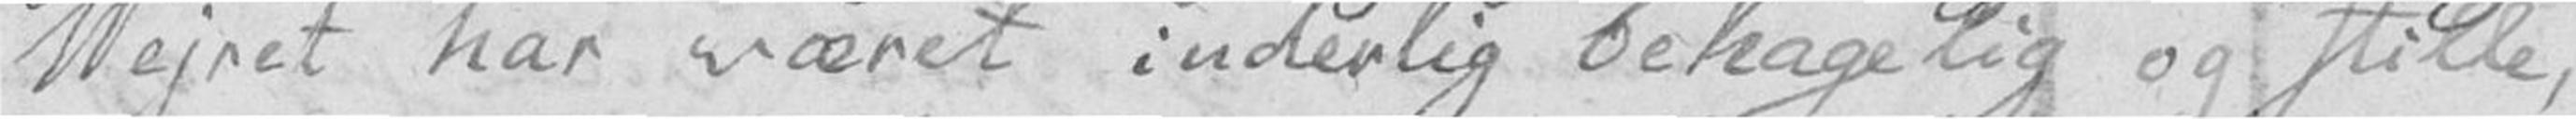Vejret har været inderlig behagelig og stille,
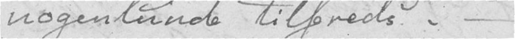nogenlunde tilfreds. –
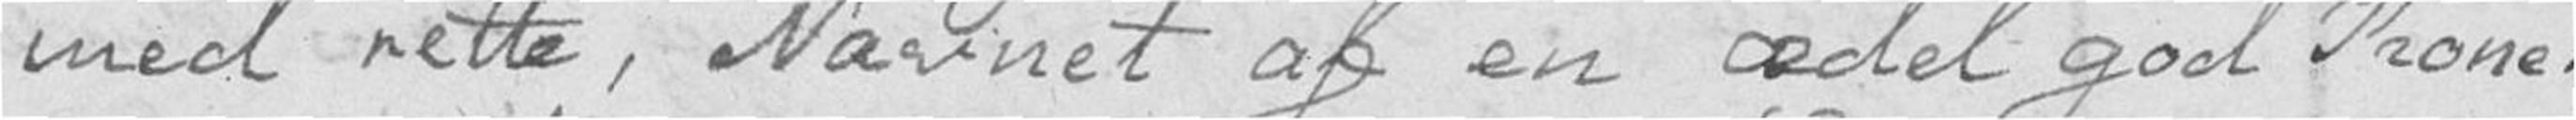med rette, Navnet af en ædel god Kone.
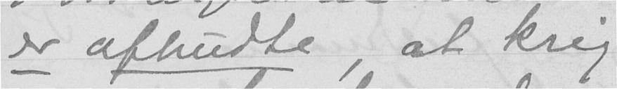er afbrudte, at krig
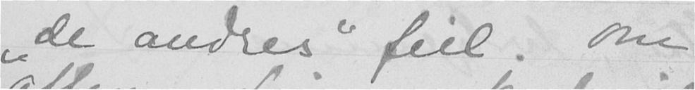"de andres" feil. Om
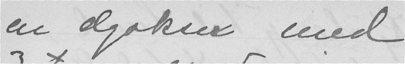en elgokse med
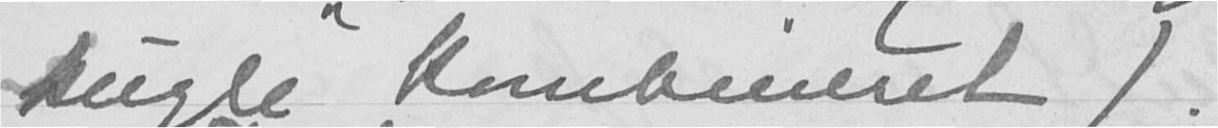kugle kombineret).
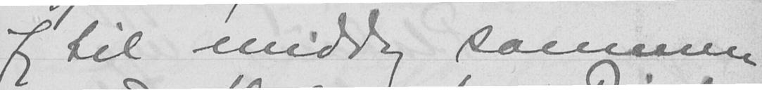Jeg til middag sammen
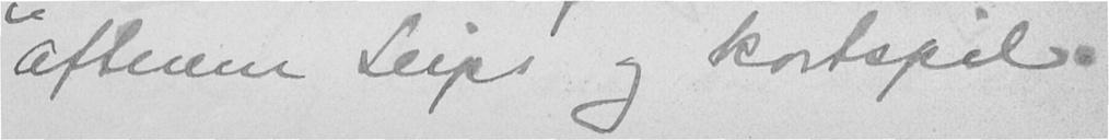aftenen Seips og kortspil.
